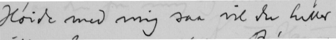Høide med mig saa vil du heller
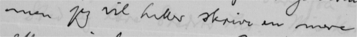men jeg vil heller skrive en mere
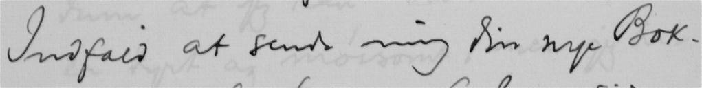Indfald at sende mig din nye Bok.
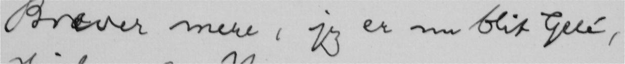Brever mere, jeg er nu blit Gelé,
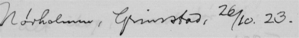Nørholmen, Grimstad, 26/10. 23.
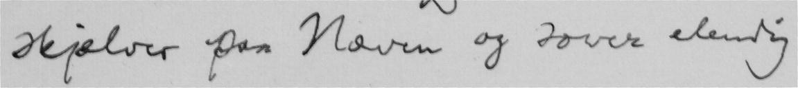skjælver paa Næven og sover elendig
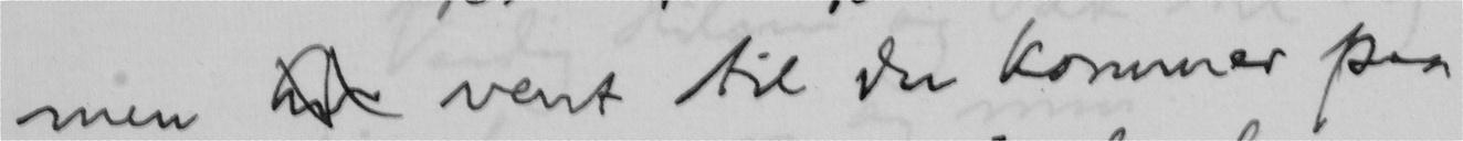men hv vent til du kommer paa
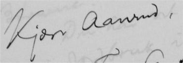Kjære Aanrud,
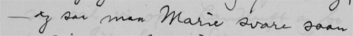- og saa maa Marie svare saan
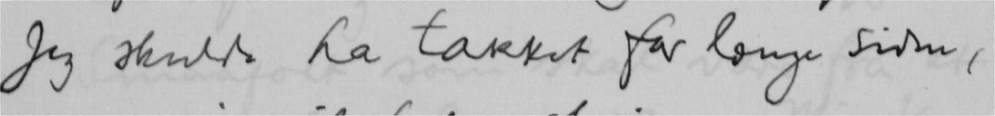Jeg skulde ha takket for længe siden,
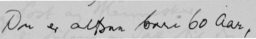Du er altsaa bare 60 Aar,
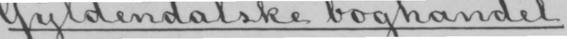Gyldendalske boghandel.
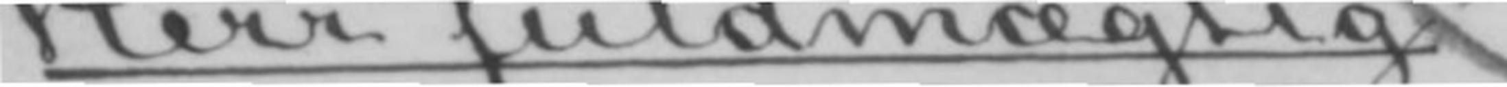Herr fuldmægtig
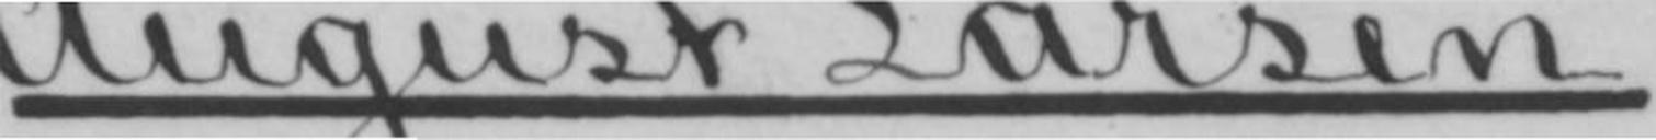August Larsen,
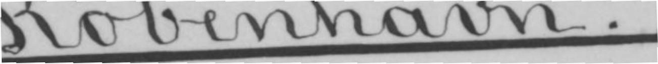København.
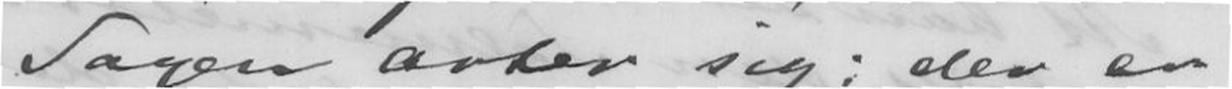Sagen arter sig; der er
